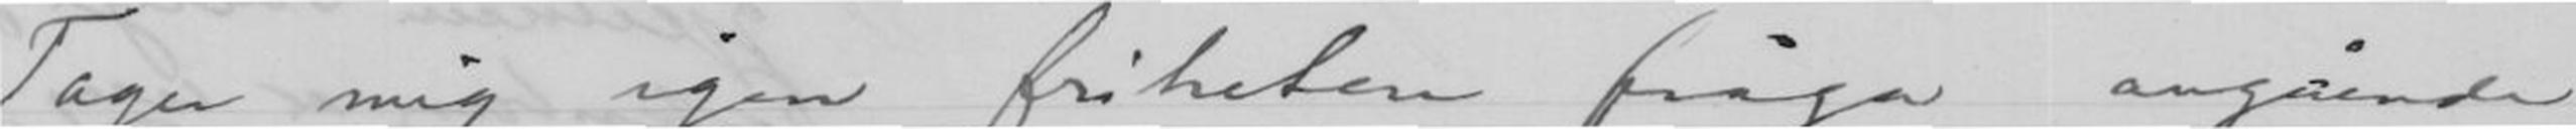Tager mig igen friheten fråga angående
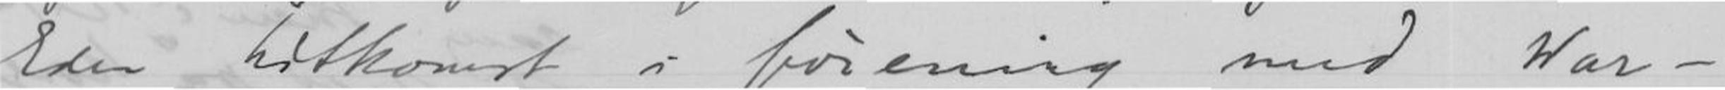Eder hitkomst i förening med War-
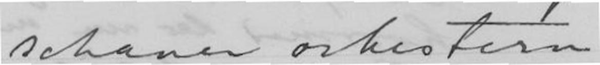schau orkestern.
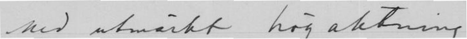Med utmärkt högaktning
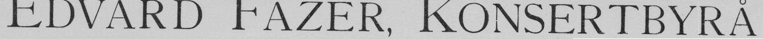EDVARD FAZER, KONSERTBYRÅ
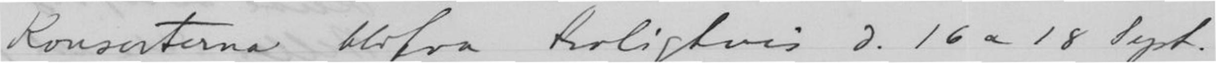Konserterna blifva troligtvis d. 16 a 18 Sept.
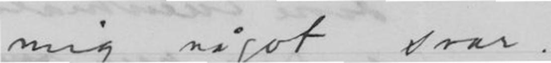mig något svar.
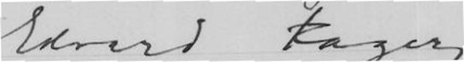Edvard Fazer
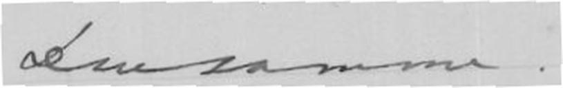Den samme.
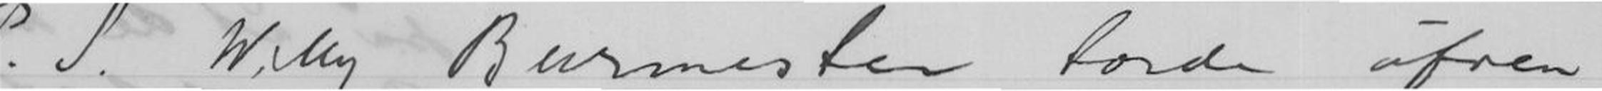P.S. Willy Burmester torde äfven
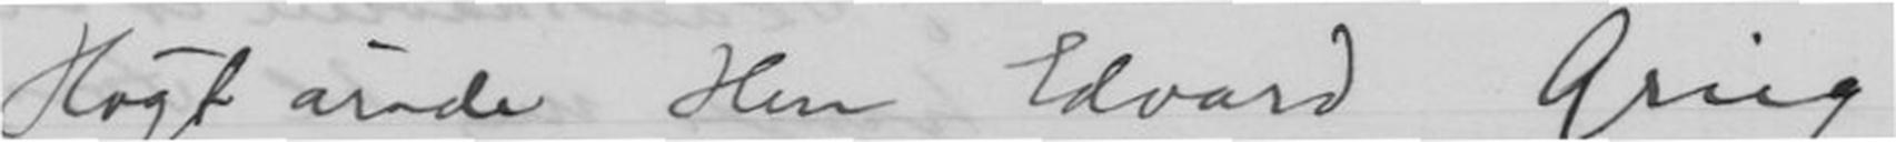Högt ärade Herr Edvard Grieg
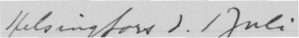Helsingfors d. 11 Juli
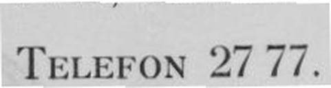TELFON 27 77.
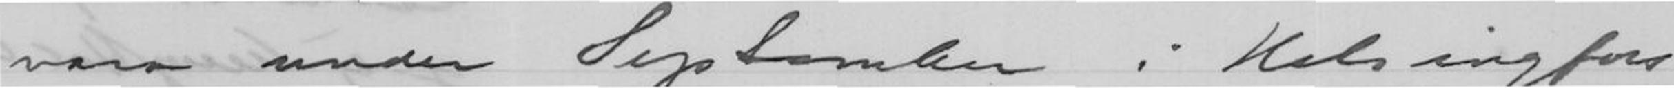vara under September i Helsingfors
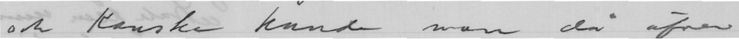och kanske kunde man då äfven
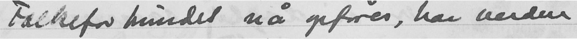Folkeforbundet nå opfører, har verden
